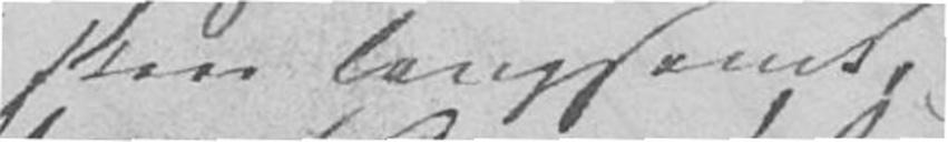skeer langsomt
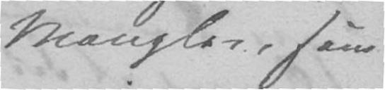Mangler, som
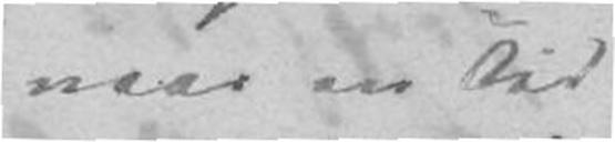naar en Tid
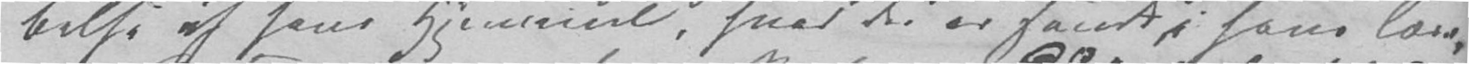belse af hans Hjemmel, hvad der er sandt i hans Lære,
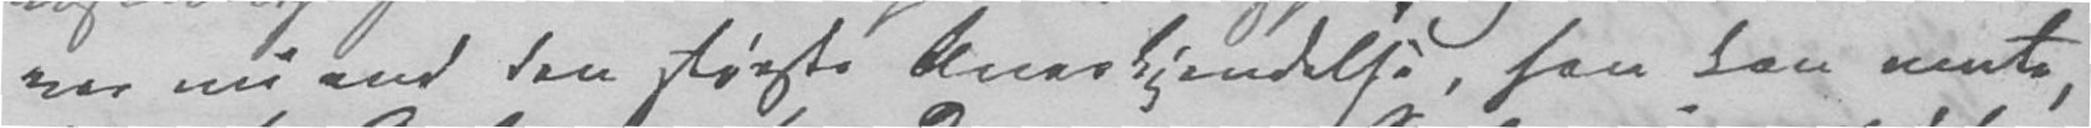ver nu end den største Anerkjendelse, han kan vente,
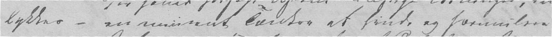lykkes – en eminent Tænker at finde og formulere
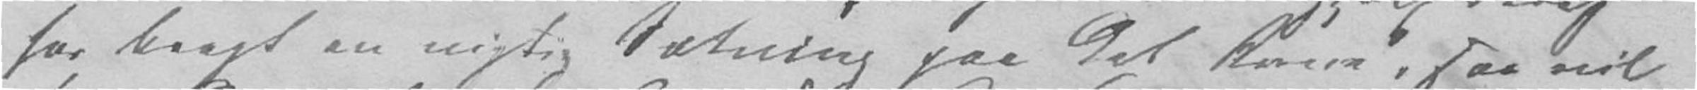har bragt en vigtig Sætning paa det Rene, saa vil
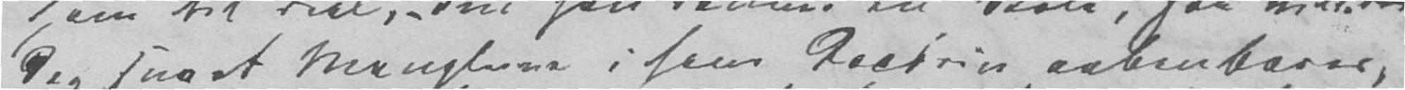dog snart Manglene i hans Doctrin aabenbares.
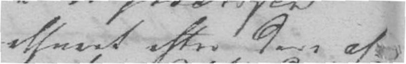ethvert efter den af-
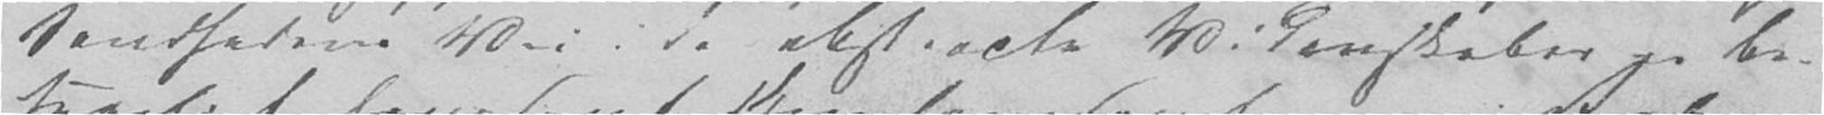Sandhedens Vei i de abstracte Videnskaber er be-
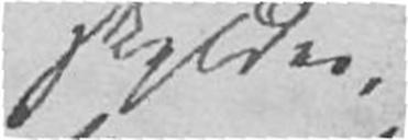skyldes,
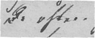De oftere
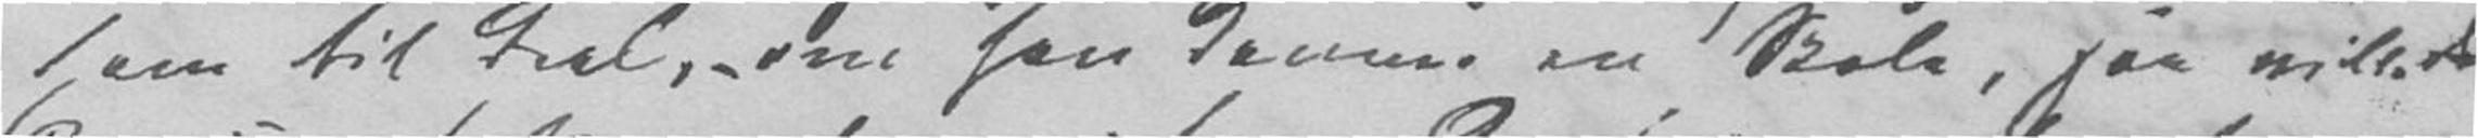ham til Deel, - om han danner en Skole, saa ville
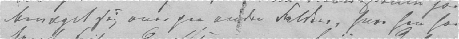bevæget sig over paa andre Feldter, hvor han har
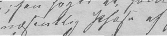væsentlig Phase af
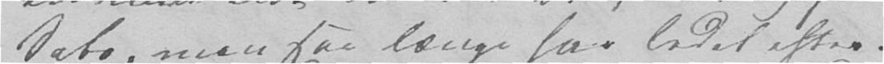Sats, man saa længe have ledet efter.
